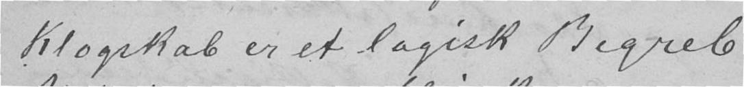Klogskab er et logisk Begreb
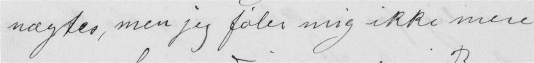nægtes, men jeg føler mig ikke mere
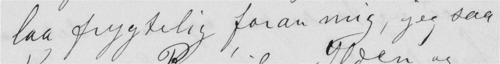laa fygtelig foran mig, jeg saa
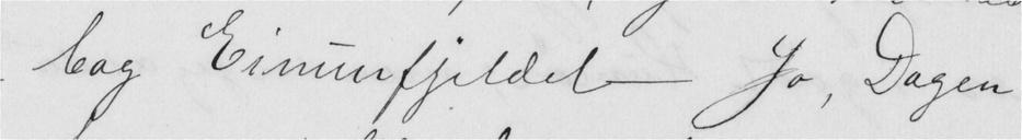bag Eimenfjeldet - Jo, Dagen
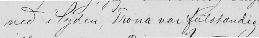ned i Syden, Trona var fulstændig
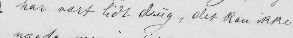har vært lidt drùg, det kan ikke
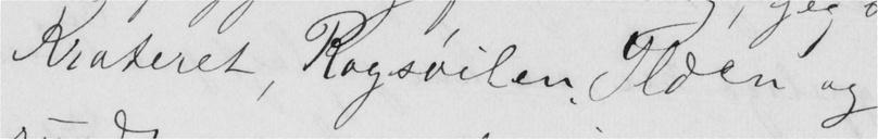Krateret, Rogsvilen Ilden og
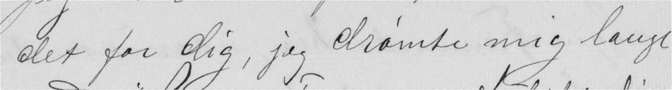det for dig, jeg drømte mig langt
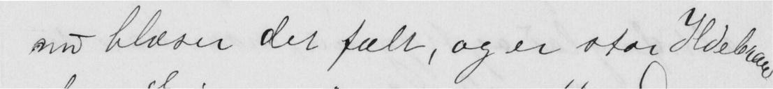nu bliver det fælt, og en stor Ildebrand
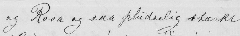og Rosa og saa pludselig stærke
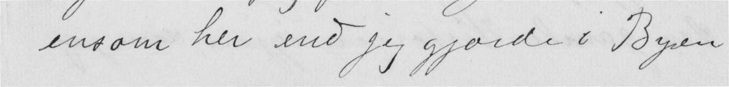ensom her end jeg gjorde i Byen
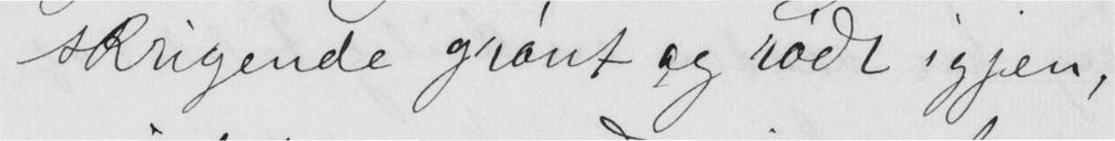skrigende grønt og rødt igjen,
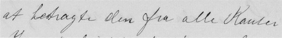at betragte den fra alle Kanter
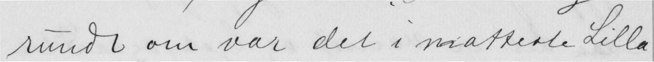rundt om var det i matteste Lilla
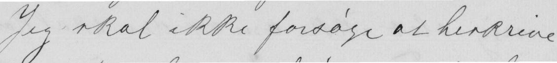Jeg skal ikke forsøge at herkreve
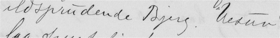ildsprudende Bjerg. Vedev
